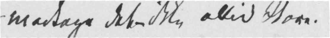modtage det ikke altid Store.
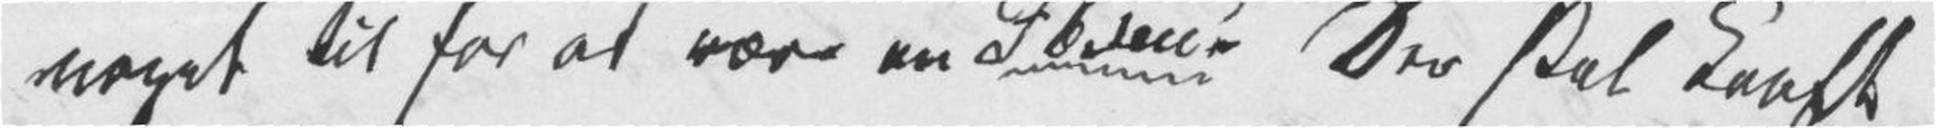noget til for at være en Ibsen! Der skal Kraft
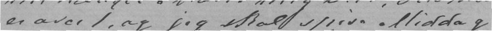er over 1 og jeg skal spise Middag
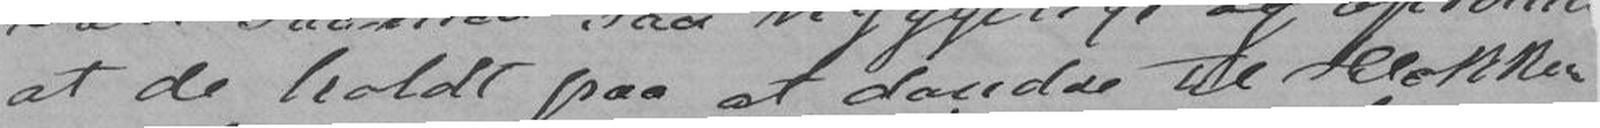at de holdt paa at dandse til Klokken
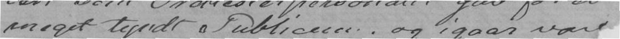meget tyndt Publicum, og igaar vare
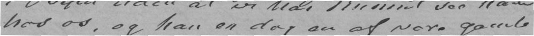hos os, og han er dog en af vore gamle
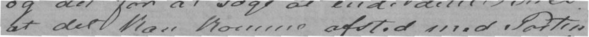at det kan komme afsted med Posten
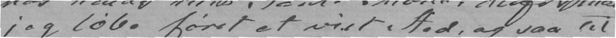jeg løbe først et vist Sted, og saa til
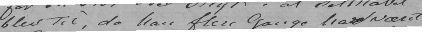blev til, da han flere Gange har været
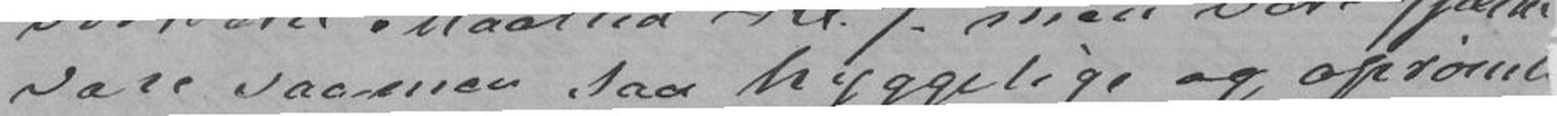vare saamen saa hyggelige og oprømt
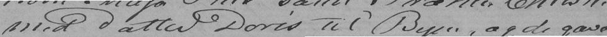med Datter Doris til Byen, og de gave
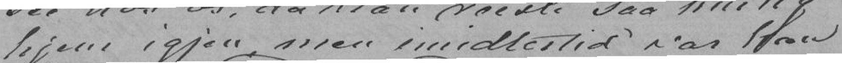hjem igjen, men imidlertid var han
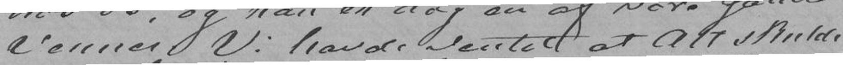Venner. Vi havde ventet at Alt skulde
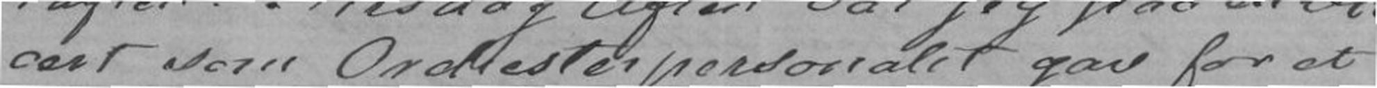cert som Orchesterpersonalet gav for et
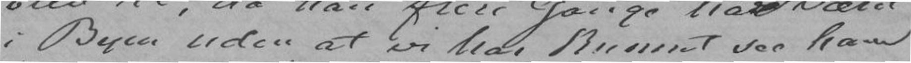i Byen uden at vi har kunnet see ham
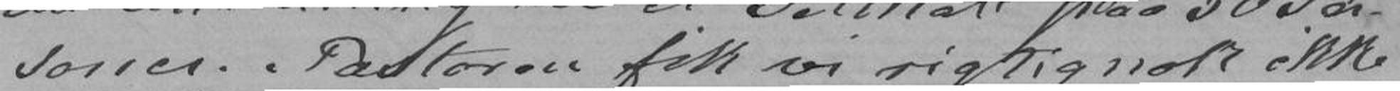soner. Pastoren fik vi rigtignok ikke
